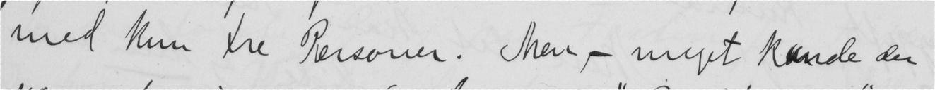med kun tre Personer. Men - meget kunde der
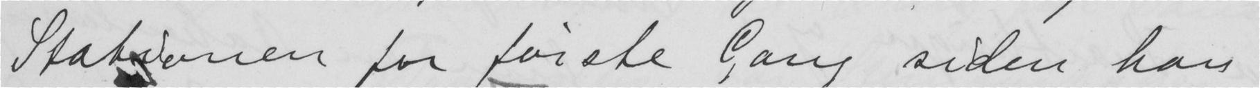Stationen for første Gang siden han
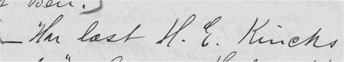- Har læst H.E. Kincks
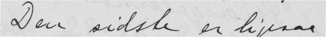Den sidste er ligesaa
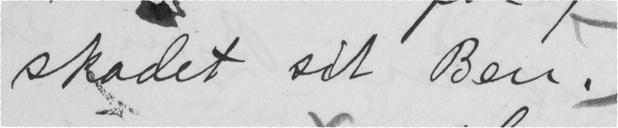skadet sit Ben.
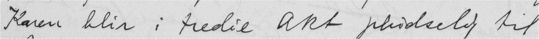Karen blir i tredie Akt pludselig til
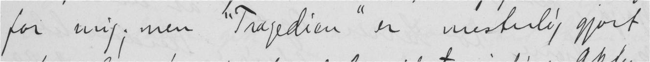for mig; men "Tragedien" er mesterlig gjort
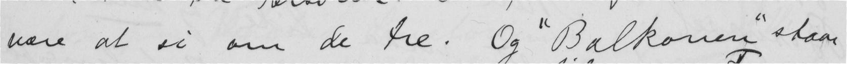være at si om de tre. Og "Balkonen" staar
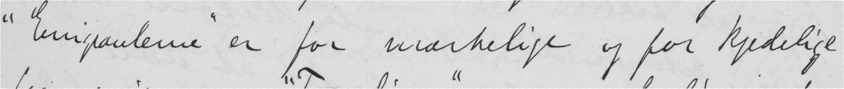"Emigrantene" er for mærkelige og for kjedelige
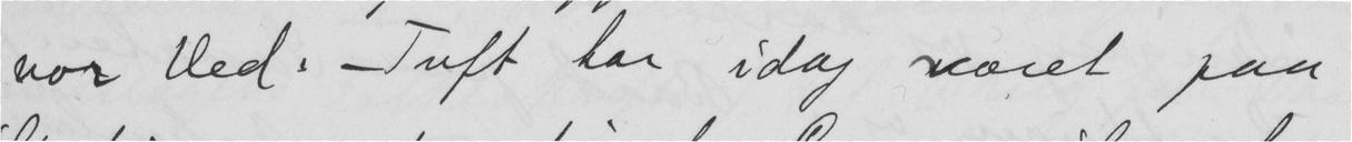vor ved. - Tuft har idag været paa
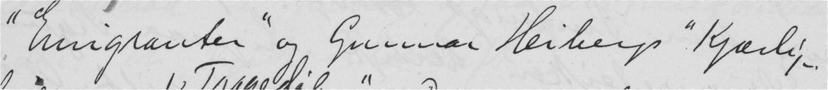"Emigranter" og Gunnar Heibergs "Kjærlig-
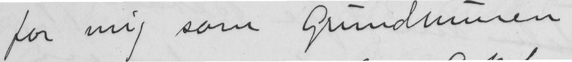for mig som Grundmuren
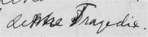denne Tragedie.
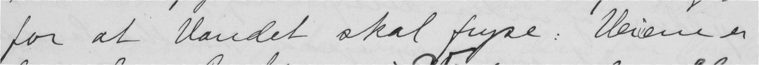for at Vandet skal fryse; Veiene er
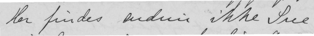Her findes endnu ikke Sne
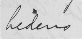hedens
